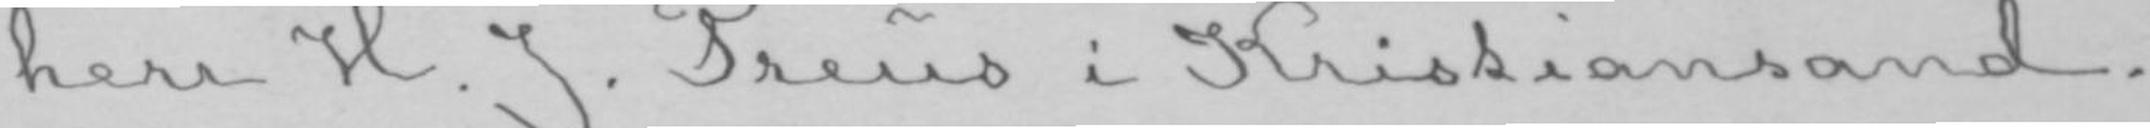herr H. J. Preus i Kristiansand.
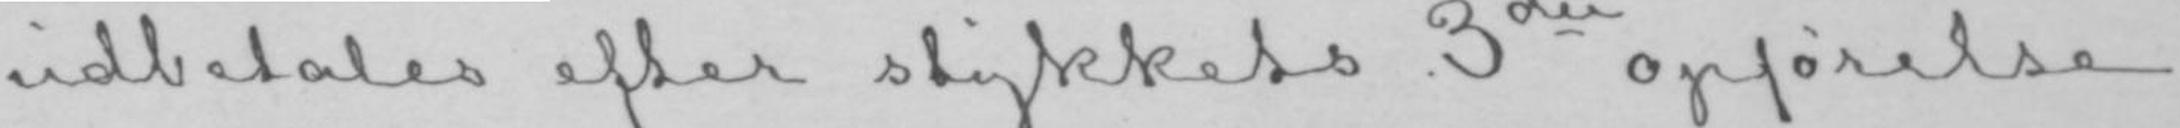udbetales efter stykkets 3die opførelse,
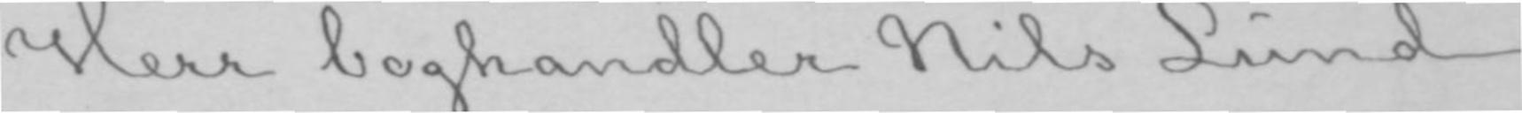Herr boghandler Nils Lund
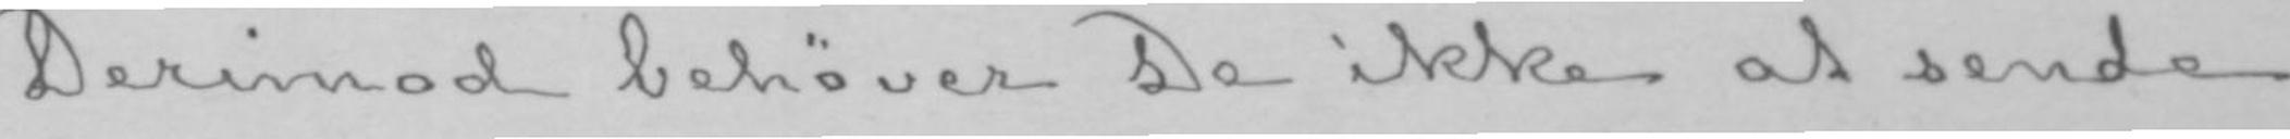Derimod behøver De ikke at sende
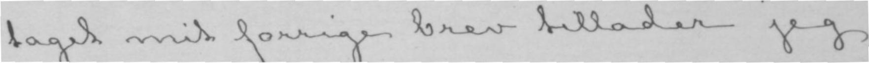taget mit forrige brev tillader jeg
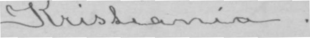Kristiania.
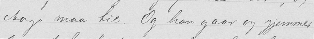Aage maa tie. Og han gaar og gjemmer
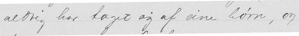aldrig har taget sig af sine børn, og
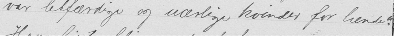var letfærdige og uærlige kvindes før hende".
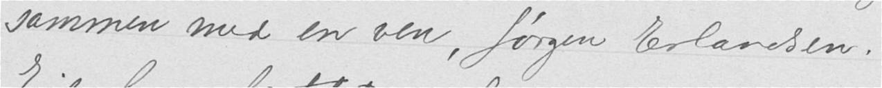sammen med en ven, Jørgen Erlandsen.
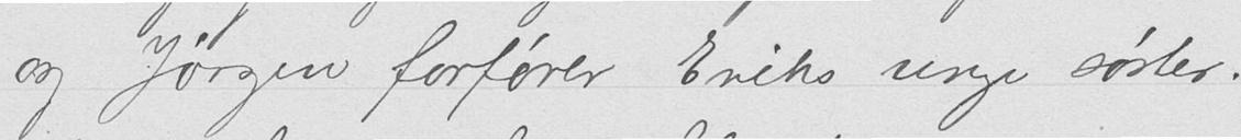og Jørgen forfører Eriks unge søster.
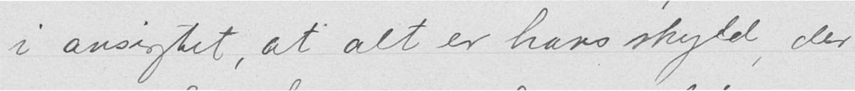i ansigtet, at alt er hans skyld, der
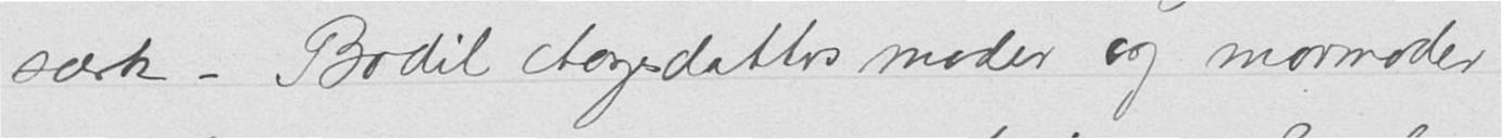særk - Bodil Aagesdatter moder og mormoder
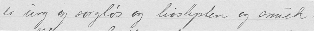er ung og sorgløs og livsleplen og smuk -
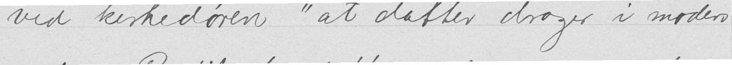ved kirkedøren "at datter drager i moders
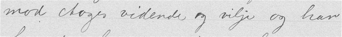mod Aages vidende og vilje og han
